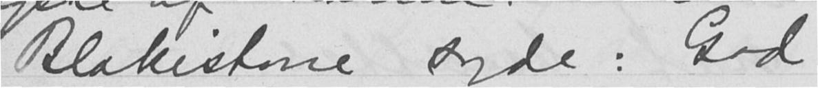Blakistone sagde: God
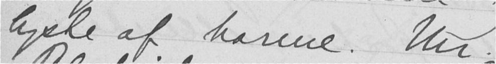lyste af harme. Mr.
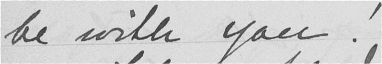be with you!
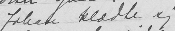Johan klædte sig
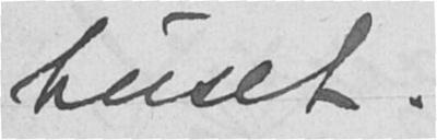huset.
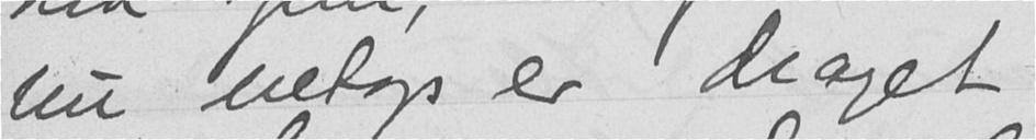nu netop er draget
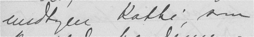undtagen Katti, som
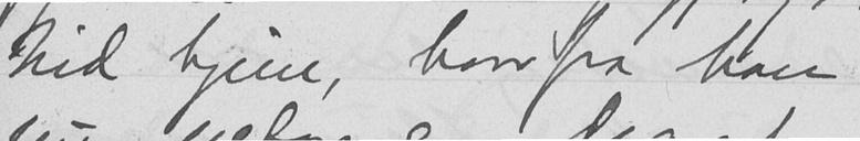hid hjem, hvorfra han
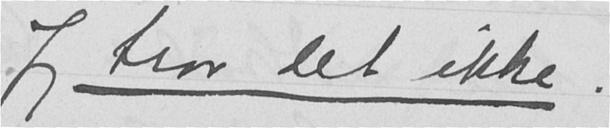Jeg tror det ikke.
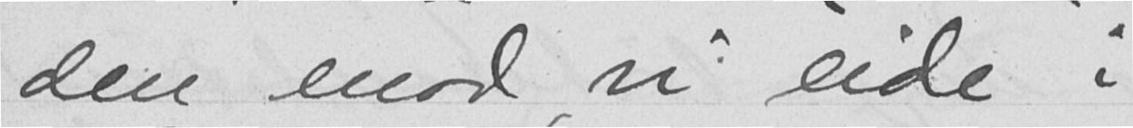den mod, vi eide i
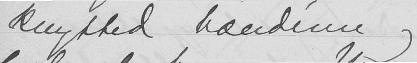knytted hænderne og
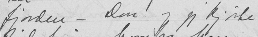fjorden - Don og jeg kjørte
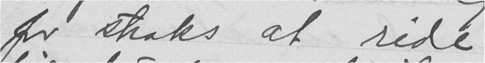for straks at ride
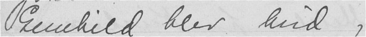Gunhild blev hvid og
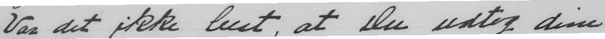Var det ikke best, at Du udtog dine
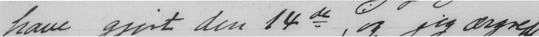have gjort den 14de, og jeg ærgrede
